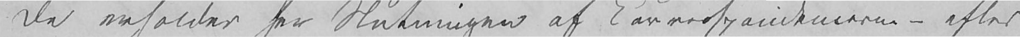De erholder her Slutningen af Korresspondencerne – efter
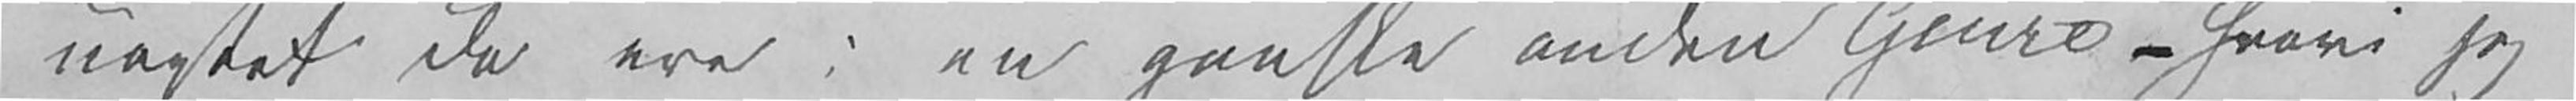uagtet de ere i en ganske anden Genre – hvori jeg
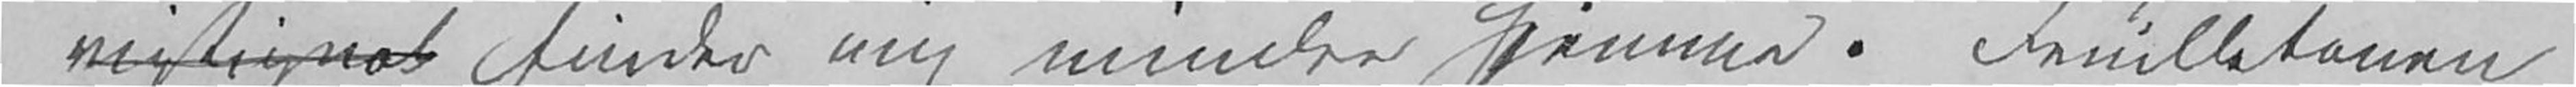rigtignok finder mig mindre hjemme. Feuilletonen
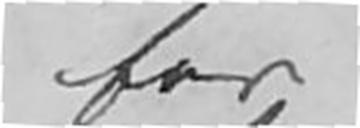for
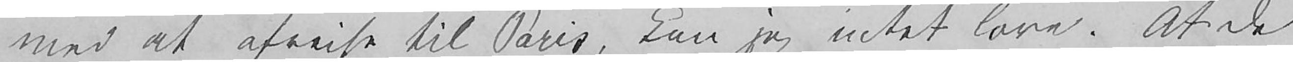med at afreise til Paris, kan jeg intet love. At De
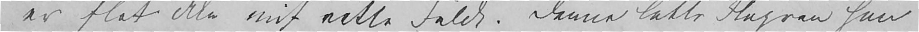er slet ikke mit rette Feldt. Denne lette Flagren hen
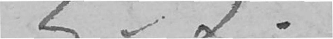C.C.
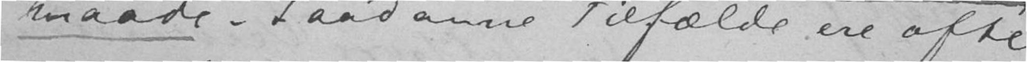maade. Saadanne Tilfælde ere ofte
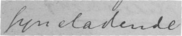syneladende
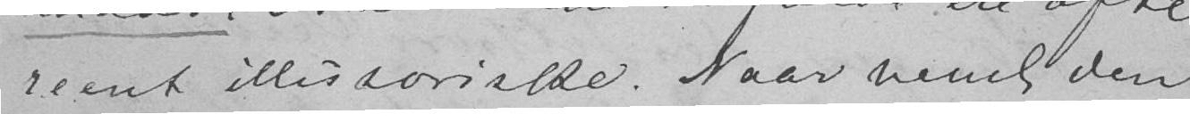reent illusoriske. Naar neml den
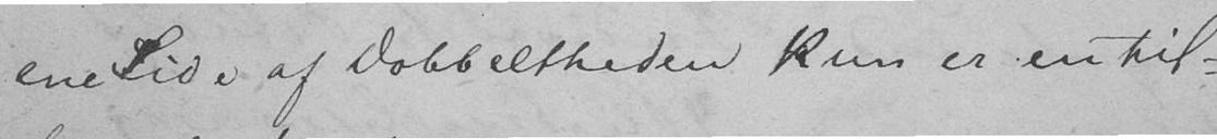ene Side af Dobbeltheden kun er en til-
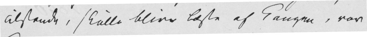Tilstande, skulle blive læste af Kongen, vor
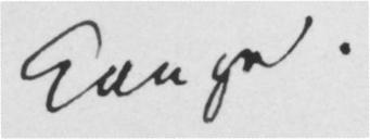Konge.
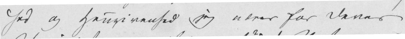og Hengivenhed jeg nærer for Deres
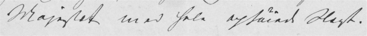Majestæt med hele ophøiede Slægt.
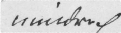mindre
